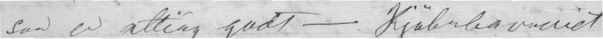saa er alting godt - Kjøbenhavneriet
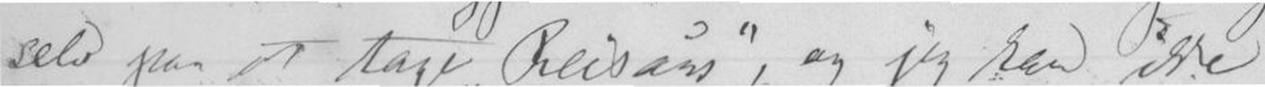selv paa at tage Reisäns, og jeg kan ikke
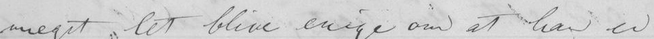meget let blive enige om at han er
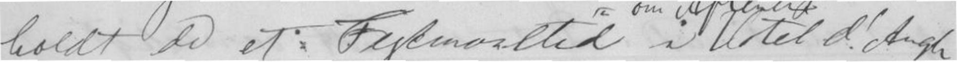holdt de et Festmaaltid i Hotel d'Angle-
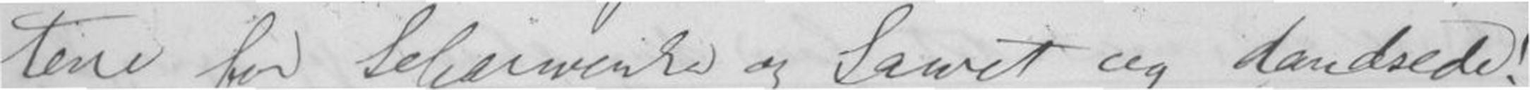tene for Scharwenska og Lauret og dandsede!
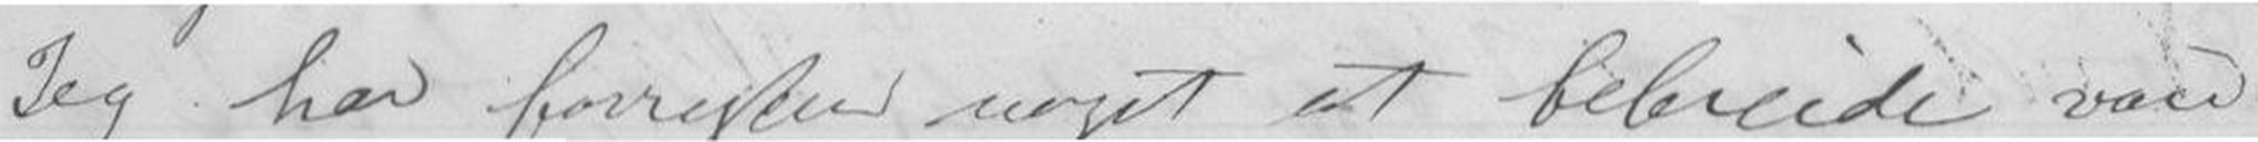Jeg har forresten noget at bebreide vaare
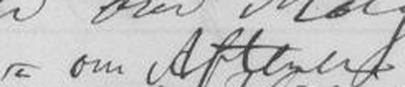om Aftenen
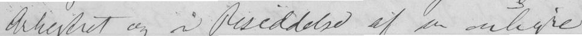Orkestret og i Besiddelse af en uhyre
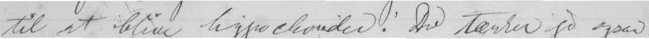til at blive hypochonder. Du tænker jo ogsaa
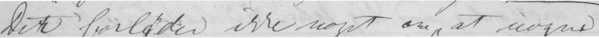Det forlyder ikke noget om, at nogen
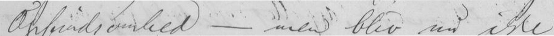Opfindsomhed - men bliv nu ikke
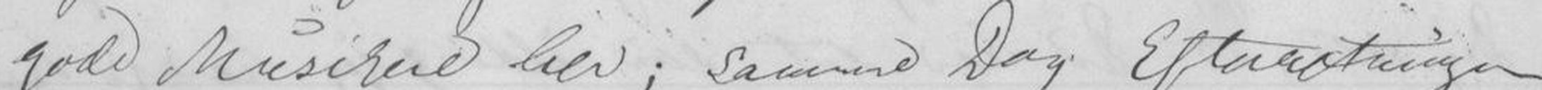gode Musikere her; samme Dag Efterretningen
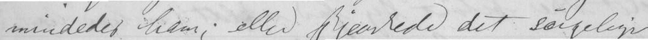mindedes ham; eller skjenkede det sørgelige
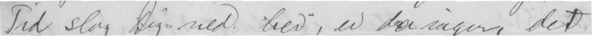Tid slog Dig ned her, er der ingen, det
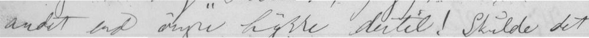andet end ønske Lykke dertil! Skulde det
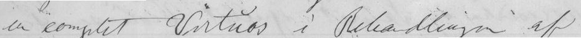en complet Virtuos i Behandlingen af
